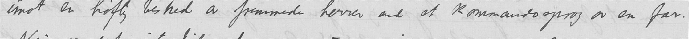imot en høflig besked av fremmede herrer end et kommandosprog av en far.
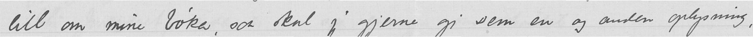litt om mine bøker, saa skal jeg gjerne gi Dem en og anden oplysning,
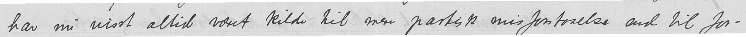har nu visst altid været kilde til mere partisk misforstaaelse end til for-
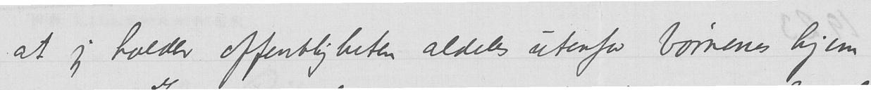at jeg holder offentligheten aldeles utenfor børnenes hjem.
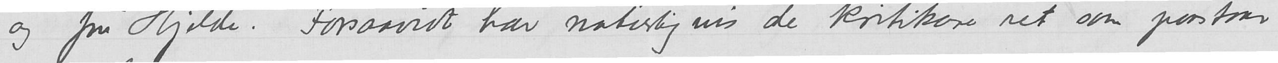og fru Hjelde. Forsaavidt har naturligvis de kritikerne ret som paastaar
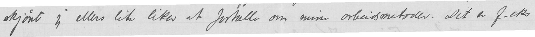skjønt jeg ellers lite liker at fortælle om mine arbeidsmetoder. Det er f.eks
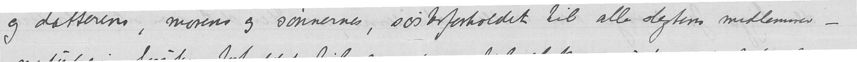og datterens, morens og sønnernes, søsterforholdet til alle slegtens medlemmer
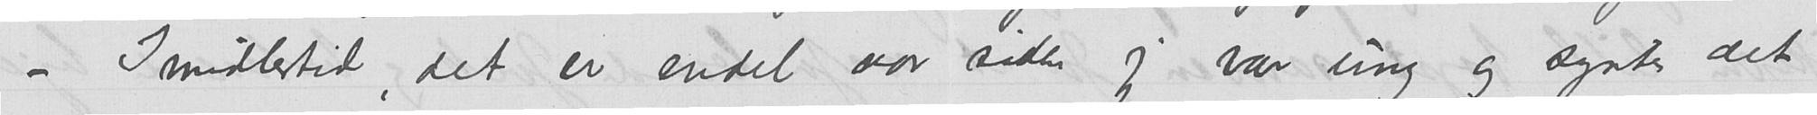– Imidlertid, det er en del aar siden jeg var ung og syntes det
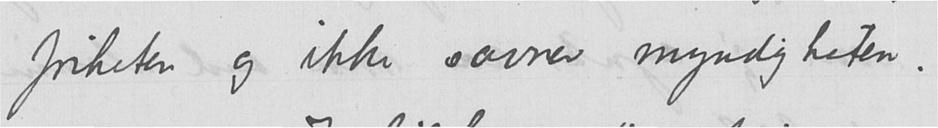friheten og ikke savner myndigheten.
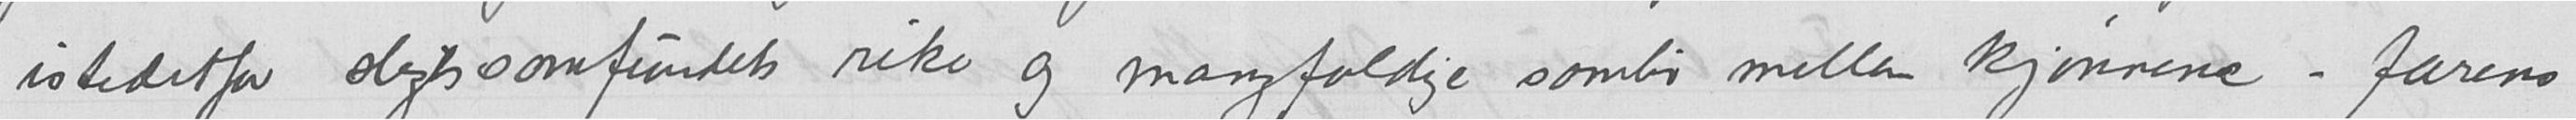istedetfor slegtssamfundets rike og mangfoldige samliv mellem kjønnene – farens
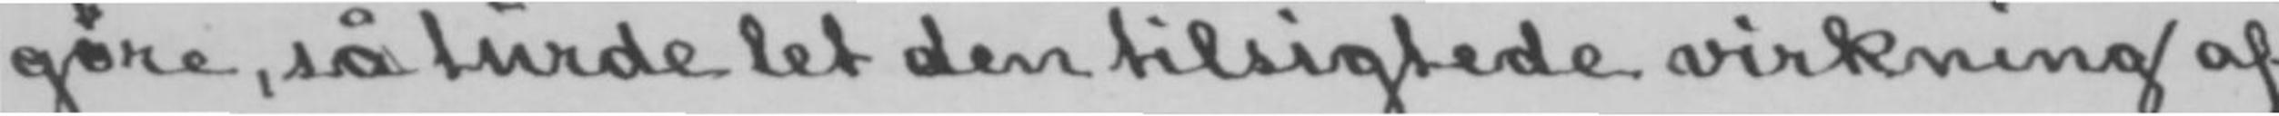gøre, så turde let den tilsigtede virkning af
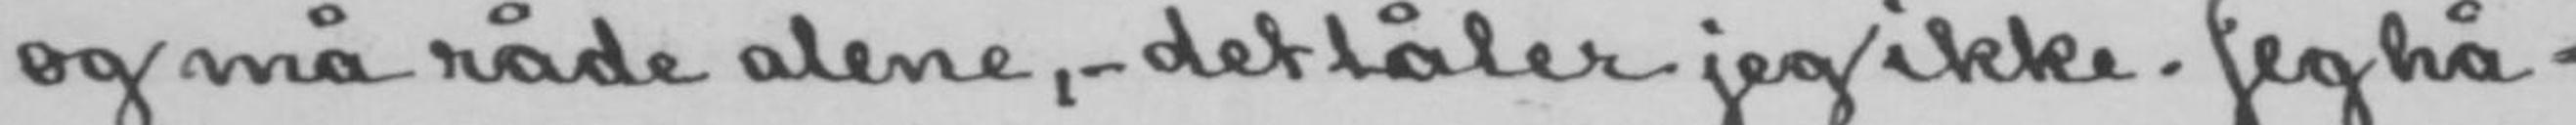og må råde alene,- det tåler jeg ikke. Jeg hå-
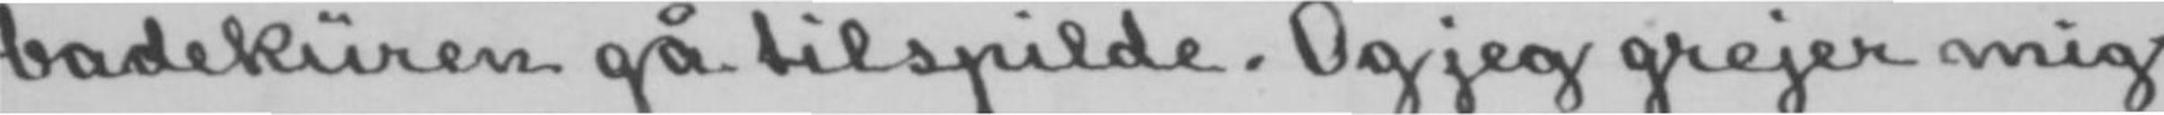badekuren gå tilspilde. Og jeg grejer mig
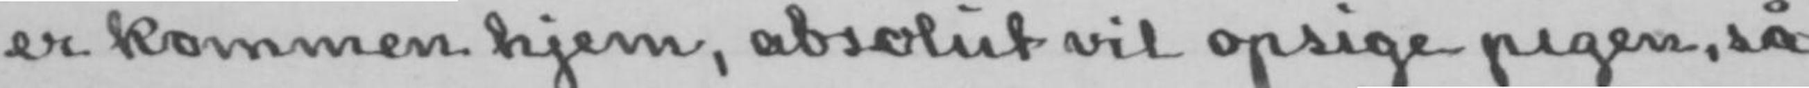er kommen hjem, absolut vil opsige pigen, så
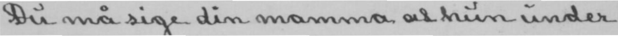Du må sige din mamma at hun under
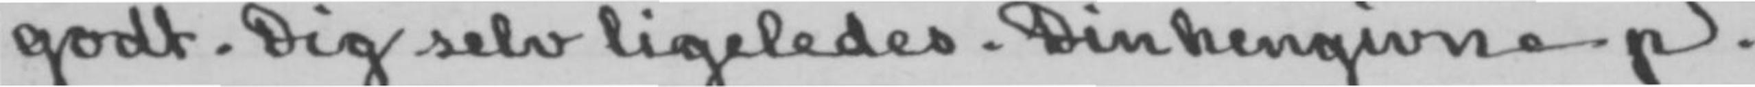godt. Dig selv ligeledes. Din hengivne p.
